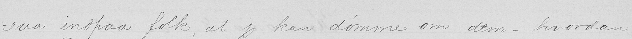saa indpaa folk, at jeg kan dømme om dem - hvordan
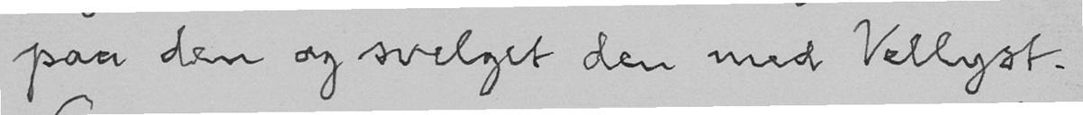paa den og svelget den med Vellyst.
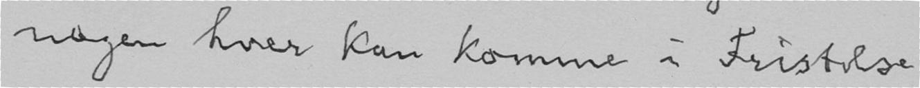nogen hver kan komme i Fristelse
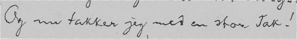Og nu takker jeg med en stor Tak!
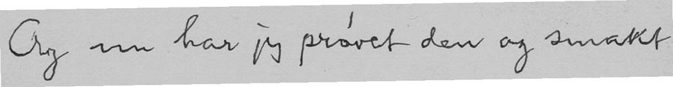Og nu har jeg prøvet den og smakt
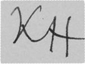KH
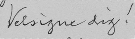Velsigne dig!
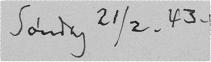Søndag 21/2. 43.
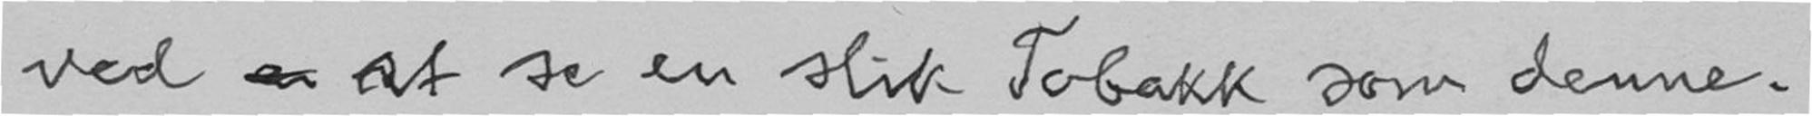ved er at se en slik Tobakk som denne.
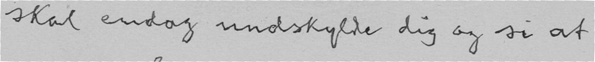skal endog undskylde dig og si at
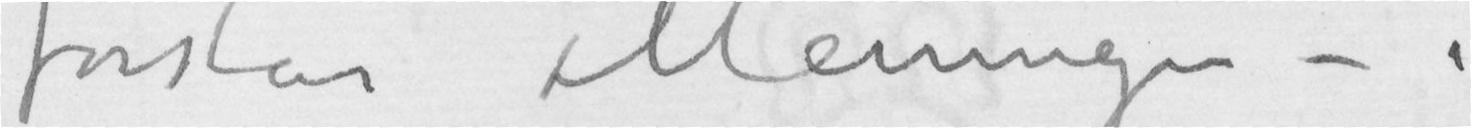forstår Meningen – i
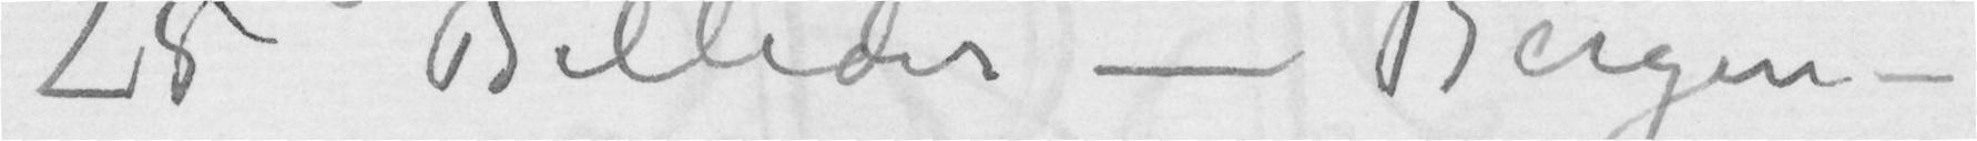25 Billeder – Bergen-
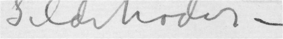Sildehoder –
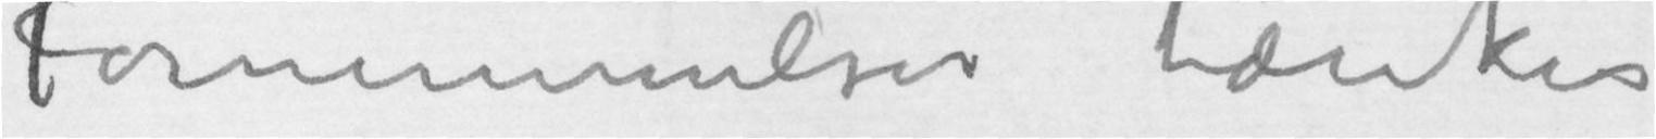Fornemmelser tænker
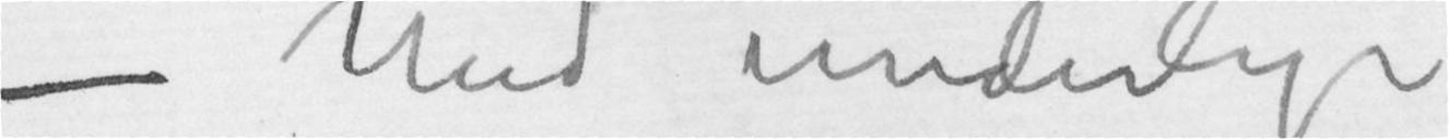– Med underlige
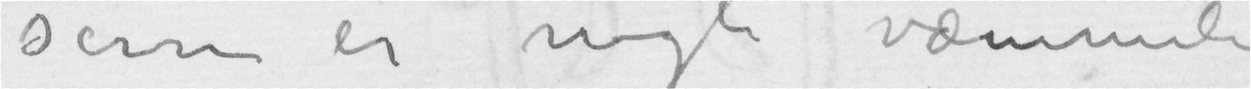sere er nogle væmmelie
